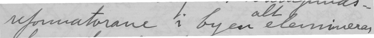reformatorane i byen alt eleminerar
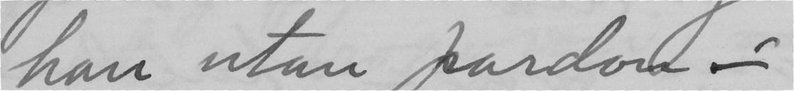han utan pardon –
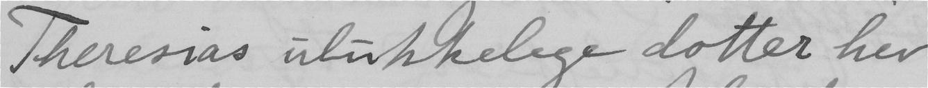Theresias ulukkelige dotter hev
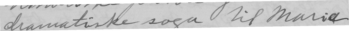dramatiske soga til Maria
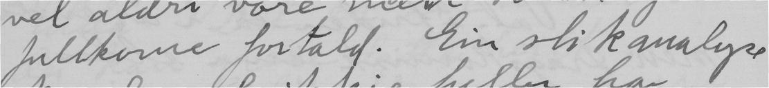fullkome fortald. Ein slik analyse
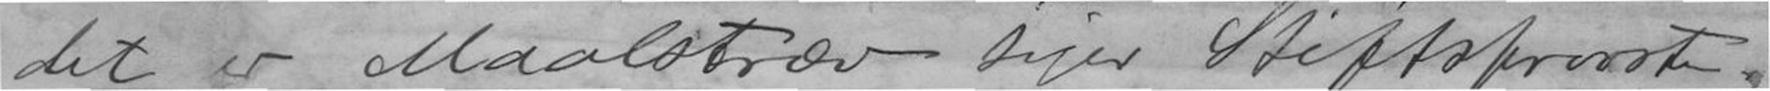det er Maalstræv siger Stiftsprovsten
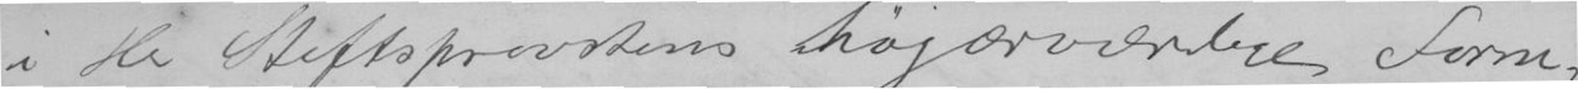i Hr Stiftsprovstens højærværdige form-
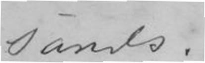sands.
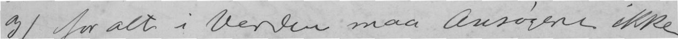3) for alt i Verden maa Ansøgere ikke
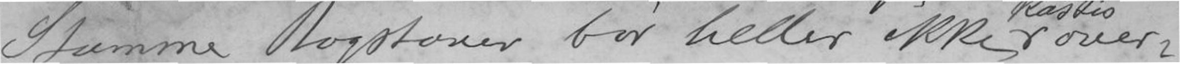Stumme Bogstaver bør heller ikke kastes over
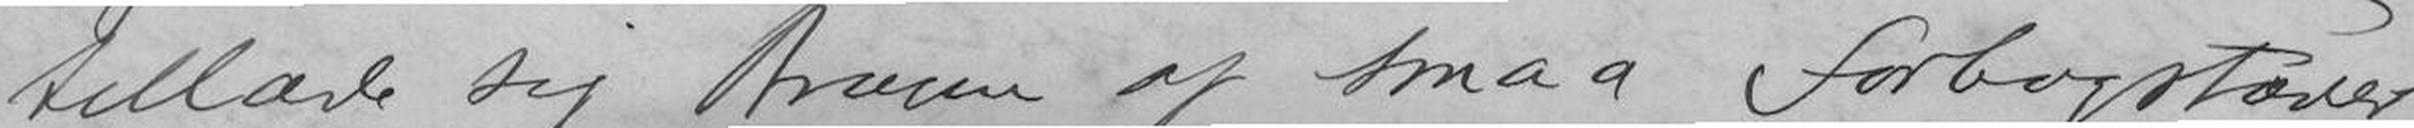tillade sig Bruken af smaa forbogstaver
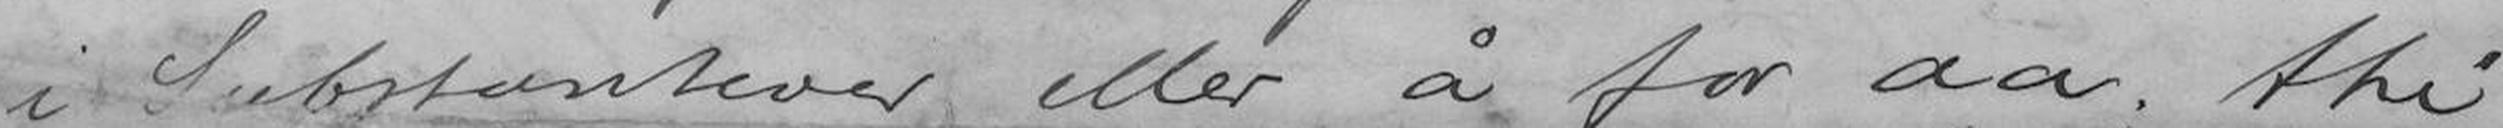i Substantiver eller å for aa; thi
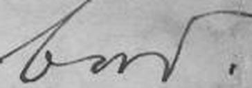bord.
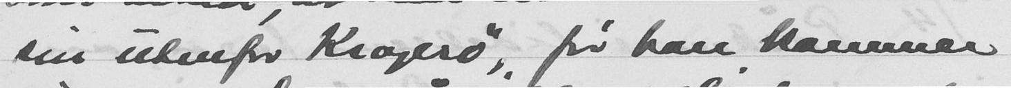sin utenfor Kragerø, før han kommer
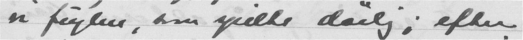vi fuglen, som spilte dårlig; efter
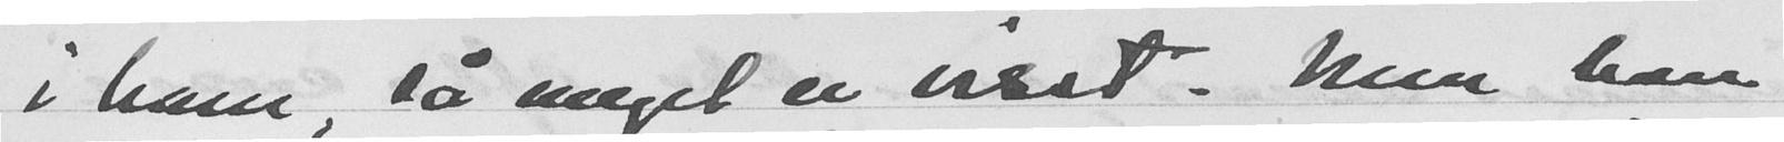i ham, så meget er visst. Men han
